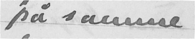på sammne
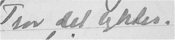Tror det lyktes.
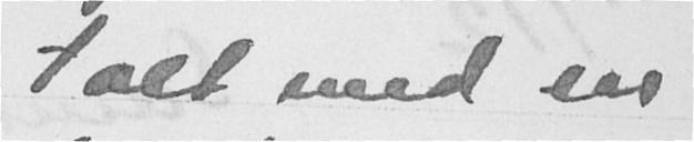Tatt med en
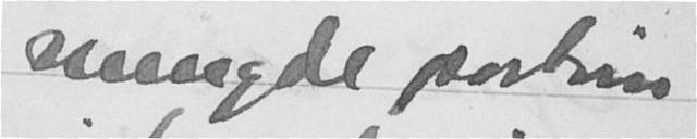mengde portion
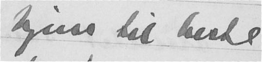hjem til berte
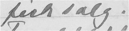fisk salg.
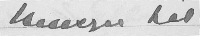Naverse dit
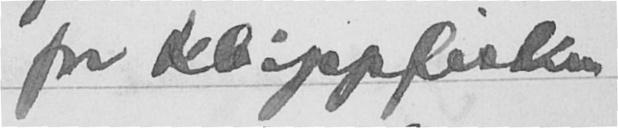for klippfisken
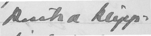Bristsa klipp-
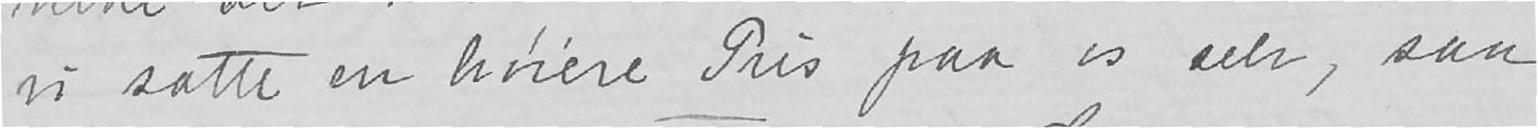vi satte en høiere Pris paa os selv, saa
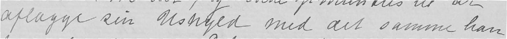aflægge sin Uskyld med det samme han
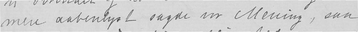mere aabenlyst sagde vor Mening, saa
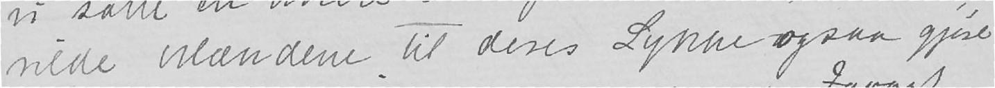vilde Mændene til deres Lykke ogsaa gjøre
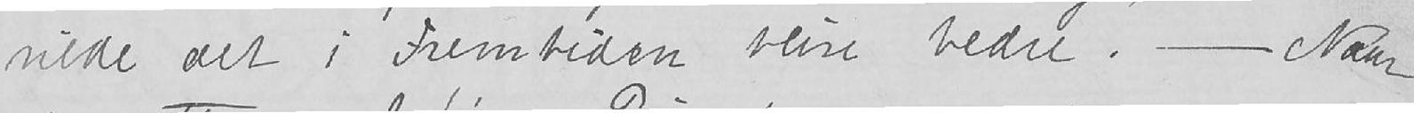vilde det i Fremtiden blive bedre. – Naar
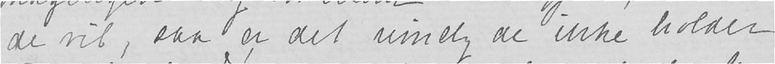de vil, saa er det rimelig de ikke holder
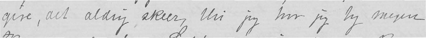give, det aldrig skeer, thi jeg tror jeg tog megen
